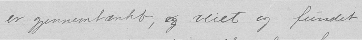er gjennomtænkt, og veiet og fundet
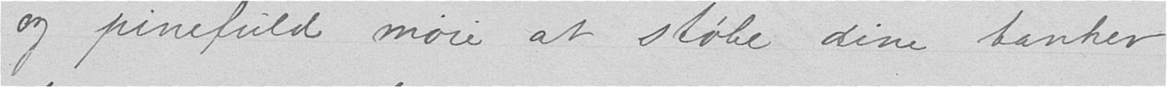og pinefuld møie at støbe dine tanker
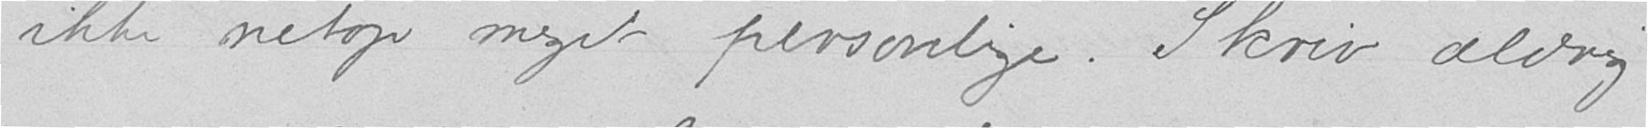ikke netop meget personlige. Skriv aldrig
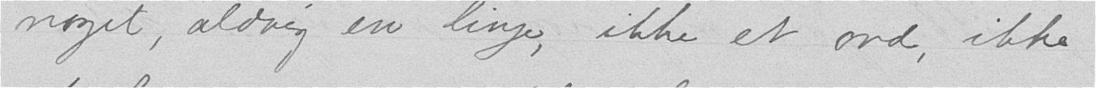noget, aldrig en linje, ikke et ord, ikke
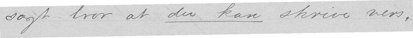sagt tror at du kan skrive vers,
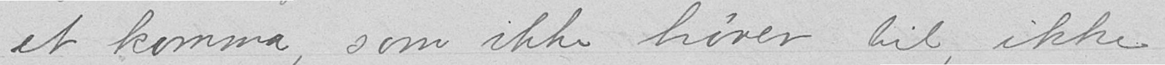et komma, som ikke hører til, ikke
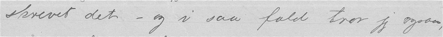skrevet det - og i saa fald tror jeg ogsaa,
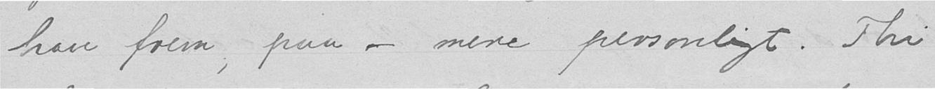have frem, paa - mere personligt. Thi
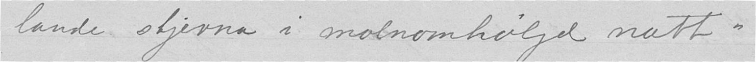lande stjerna i molnomhøljd natt"
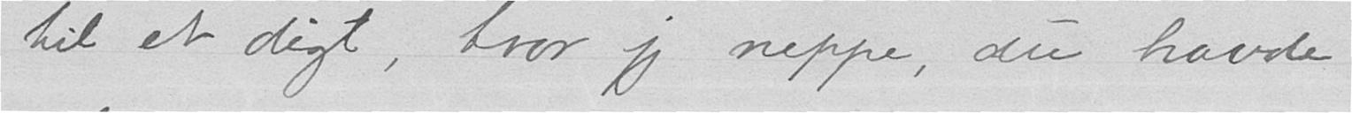til et digt, tror jeg neppe, du havde
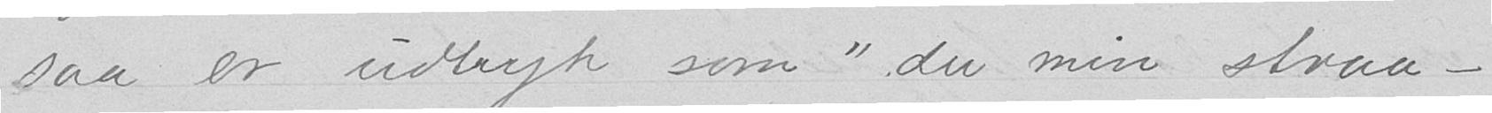saa er udtryk som "du min straa-
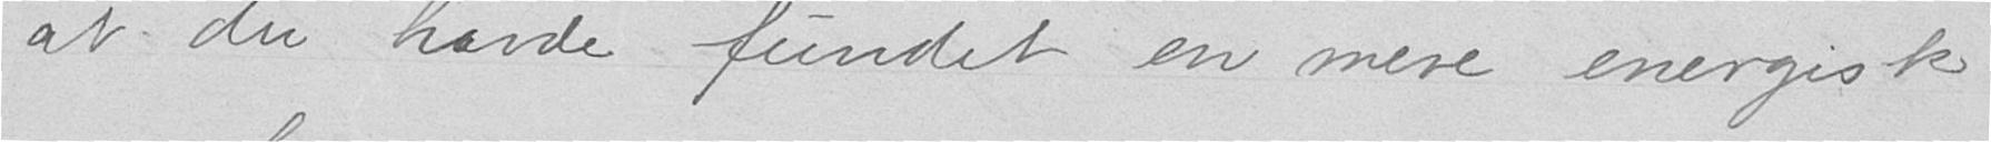at du havde fundet en mere energisk
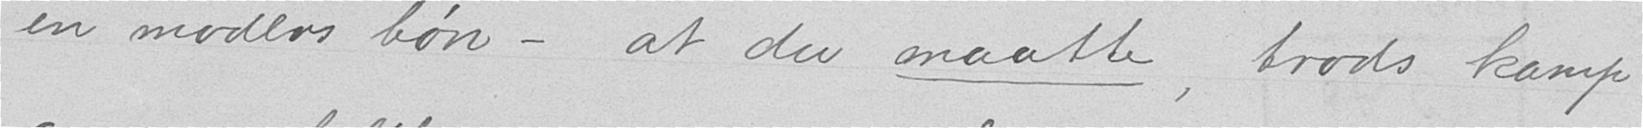en moders bøn - at du maatte, trods kamp
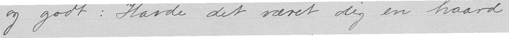og godt: Havde det været dig en haard
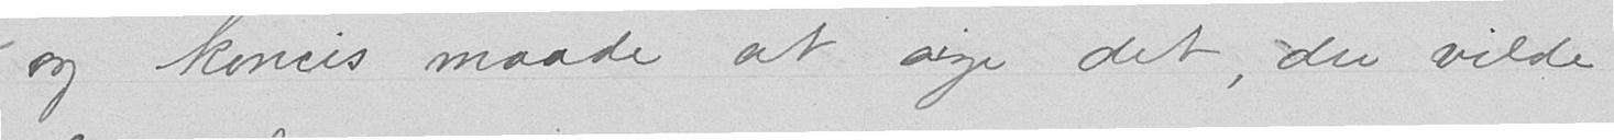og koncis maade at sige det, du vilde
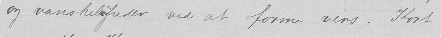og vanskeligheder ved at forme vers. Kort
